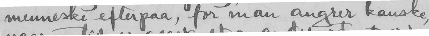menneske efterpaa, for man angrer kanske,
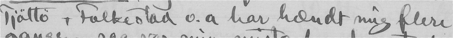Tjøttø, Folkestad o.a har hændt mig flere
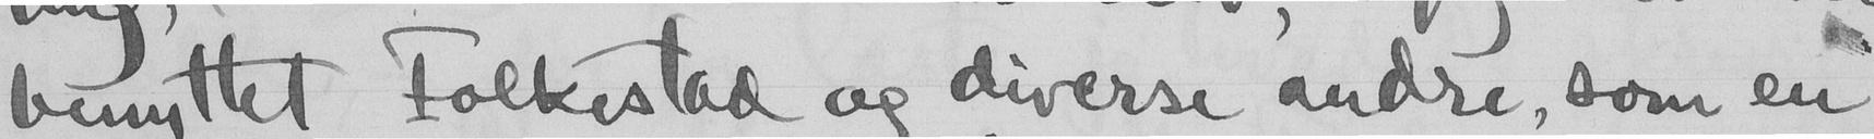benyttet Folkestad og diverse andre, som en
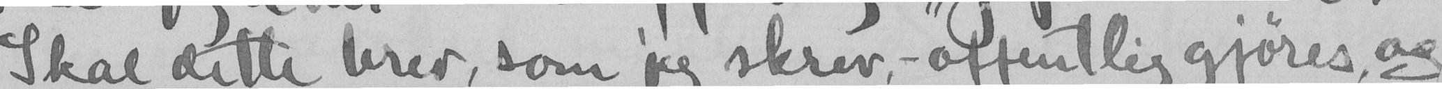Skal dette brev, som jeg skrev, - offentlig gjøres, og
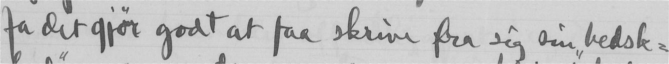Ja det gjør godt at faa skrive fra sig sin "bedsk-
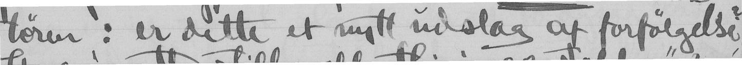tøren: er dette et nytt udslag at forfølgelse?
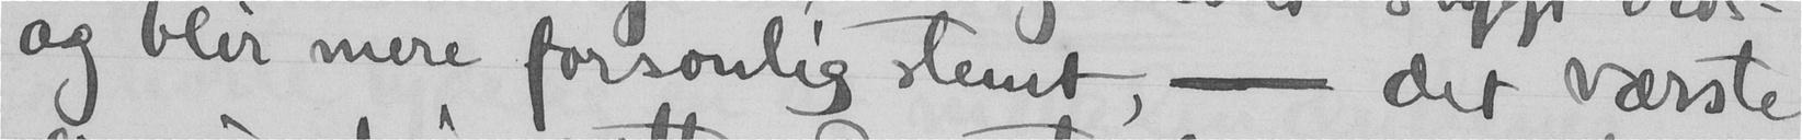og blir mere forsonlig stemt - det værste
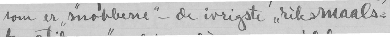som er "snobbene - de ivrigste "riksmaals-
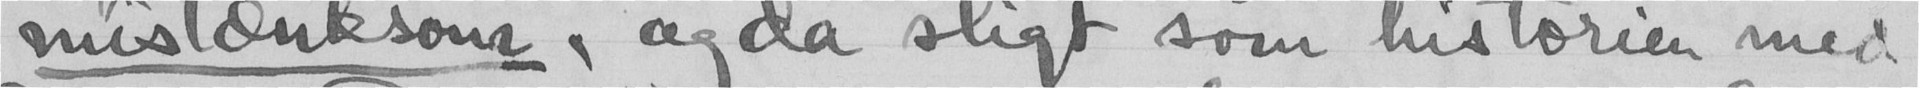mistænksom, og da sligt som historien med
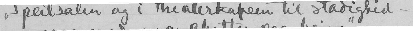Speilsalen og i Theaterkafeen til stadighed -
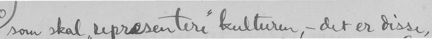som skal "repræsentere" kulturen - det er disse,
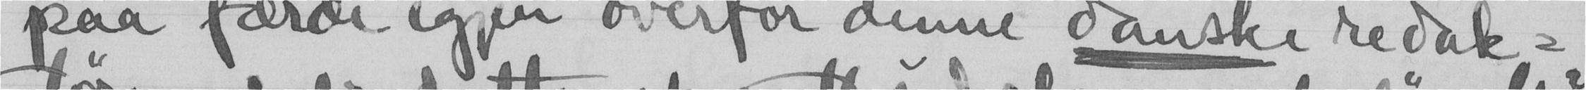paa færde igjen overfor denne danske redak-
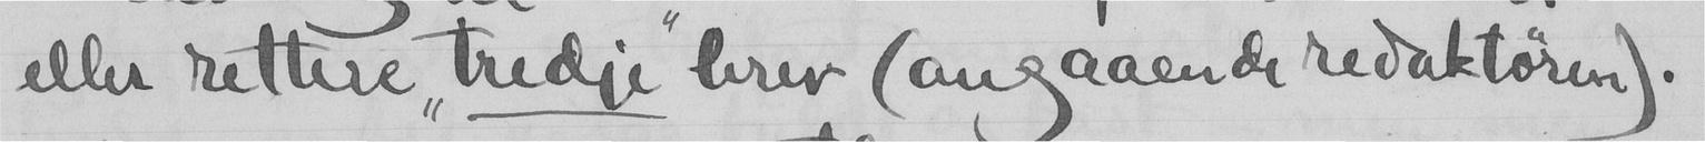eller rettere "tredje" brev (angaaende redaktøren).
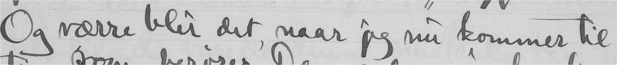Og værre blir det naar jeg nu kommer til
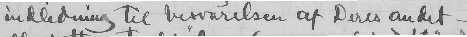indledning til besvarelsen af Deres andet -
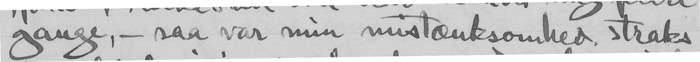gange, - saa var min mistænksomhed straks
